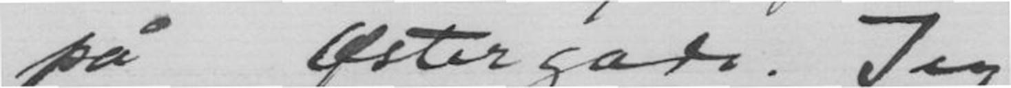på Østergade. Jeg
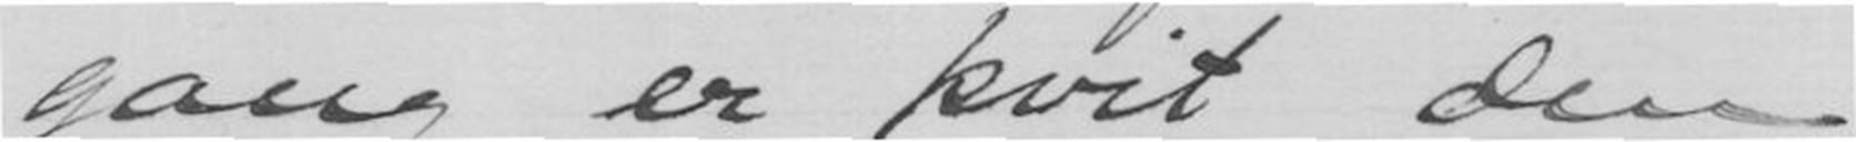gang er kvit den
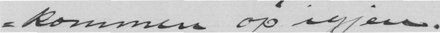kommen op igjen.
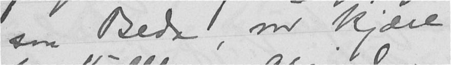saa Beda, vor kjære
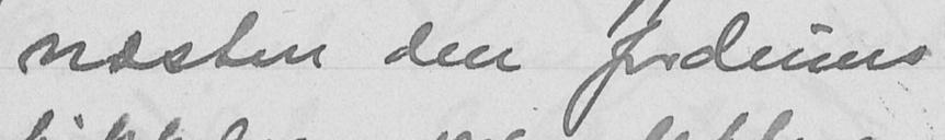næsten den fordums
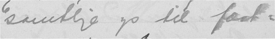samtlige op til fæst-
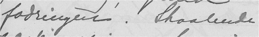fodringen. Straalende
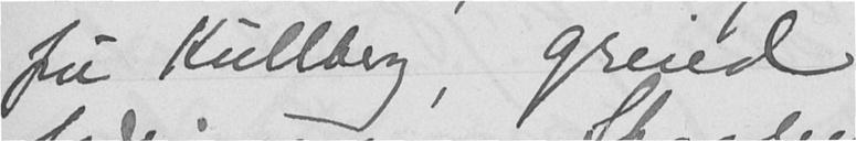fru Kullberg, greied
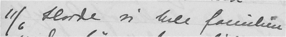11/6 Havde vi hele familien
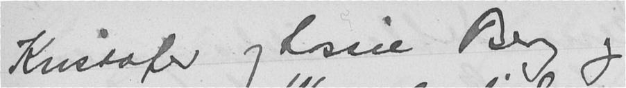Kristofer og Sossie Berg og
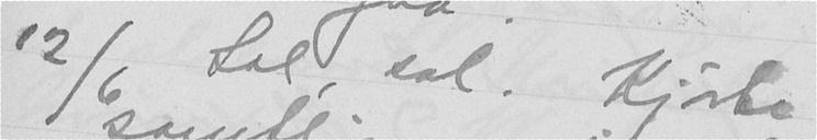12/6 Sol, sol. Kjørte
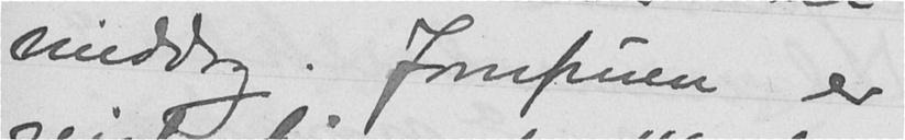middag. Jomfruen er
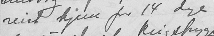mit hjem for 14 dage
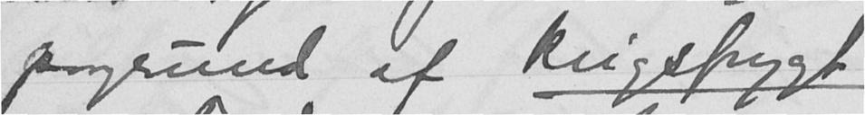paagrund af krigsfrygt,
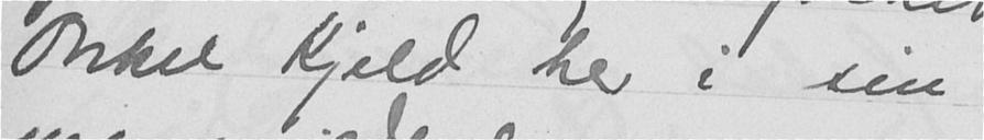Onkel Kjeld her i sin
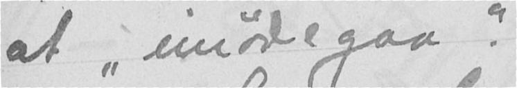at "imødegaa".
